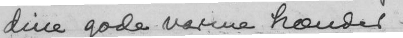dine gode varme hænder -
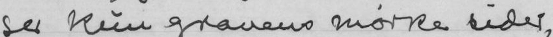ser kun gravens mørke sider,
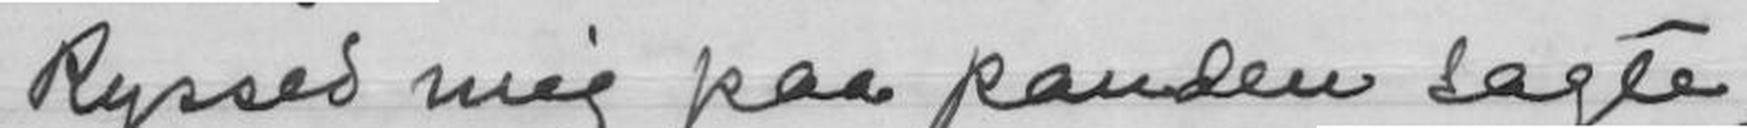Kyssed mig paa panden sagte,
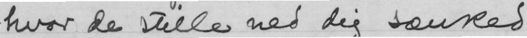hvor de stille ned dig sænked
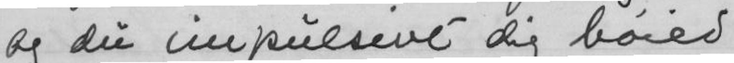Og du impulsivt dig bøied
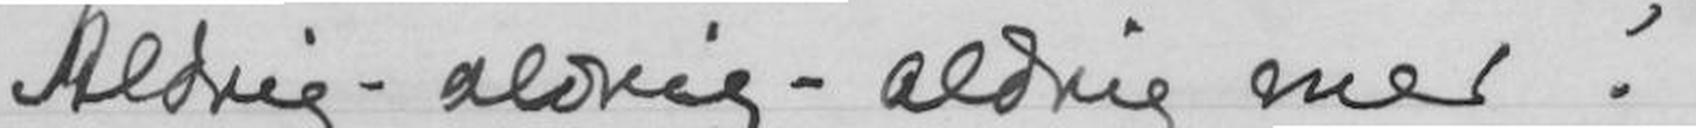Aldrig - aldrig - aldrig mer! -
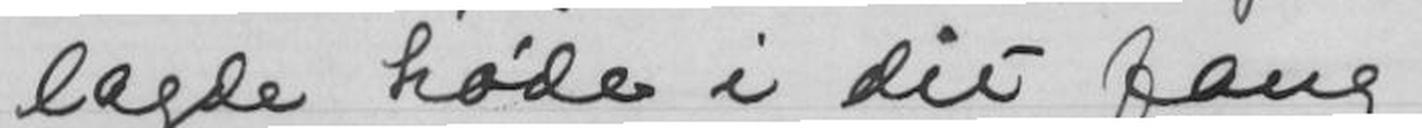lagde hode i dit fang
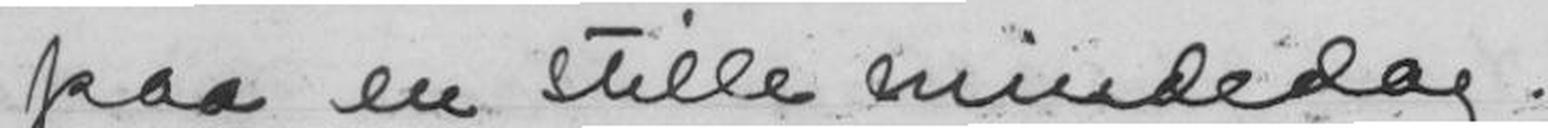paa en stille mindedag!
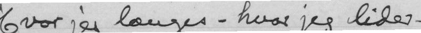Hvor jeg længes - hvor jeg lider -
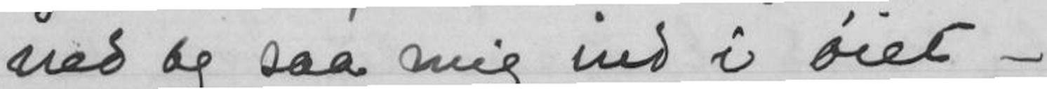ned og saa mig ind i øiet -
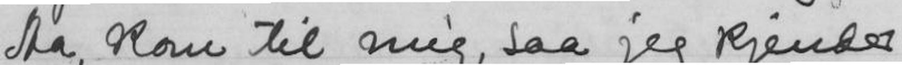Aa, kom til mig, saa jeg kjender
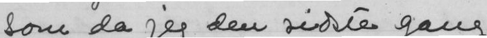Som da jeg den sidste gang
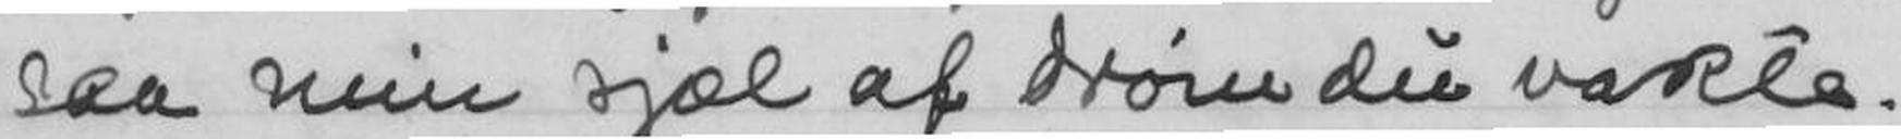saa min sjæl af drøm du vakte.
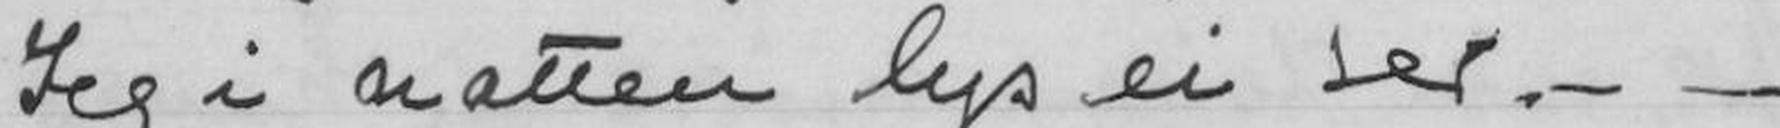Jeg i natten lys ei ser. - -
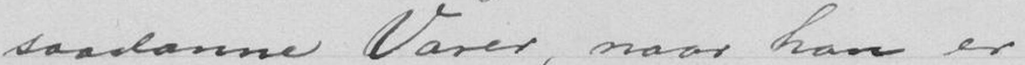saadanne Varer, naar han er
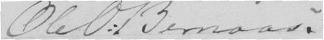Ole O:Bernaas.
